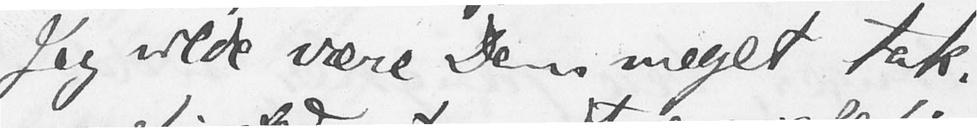Jeg vilde være Dem meget tak-
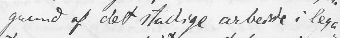grund af det stadige arbeide i lega-
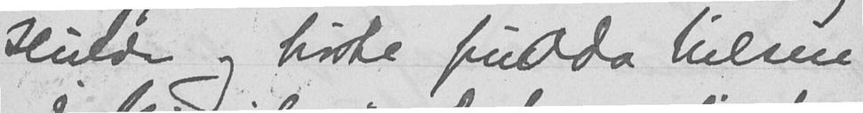Hulda og hørte fru Oda Nilsen
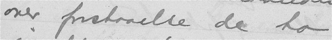over forstaaelse de to
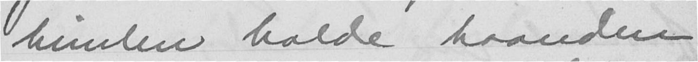himlen holde haanden
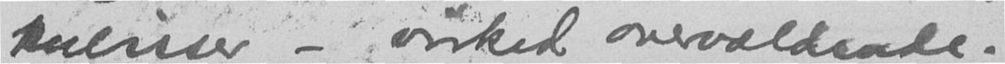kulisser - virked overvældende.
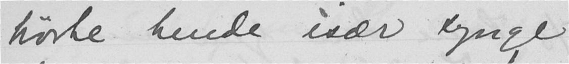hørte hende især synge
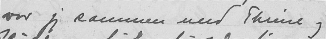var jeg sammen med Thrine og
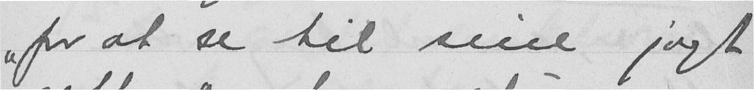"for at se til sine jagt
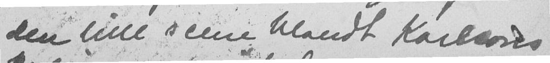den lille scene blandt Karlsons
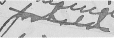fortald
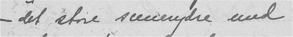- det store sceneydre med
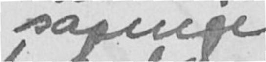sapinge
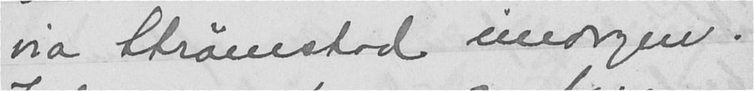via Strømstad imorgen.
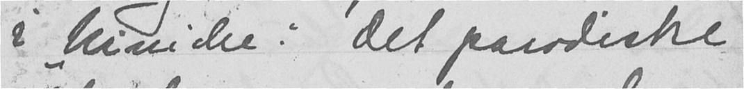i "Niniche". Det parodiske
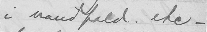i vandfald. etc -
